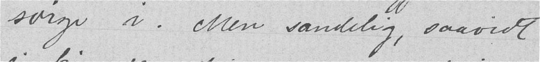sørge i. Men sandelig, saavidt
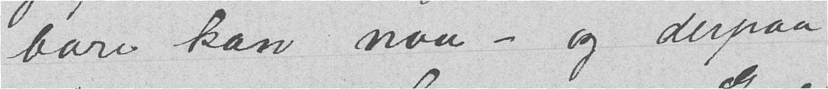bare kan naa - og derpaa
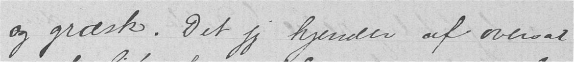og græsk. Det jeg kjender af oversat
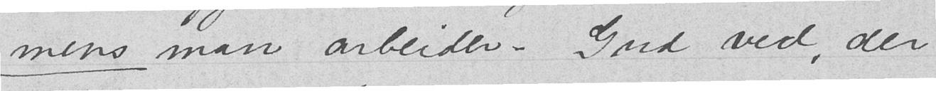mens man arbeider. Gud ved, der
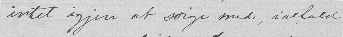intet igjen at sørge med, ialfald
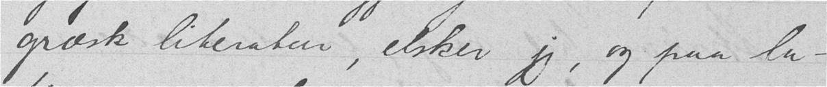græsk literatur, elsker jeg, og paa la-
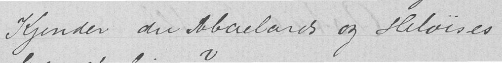Kjender du Abelards og Héloïses
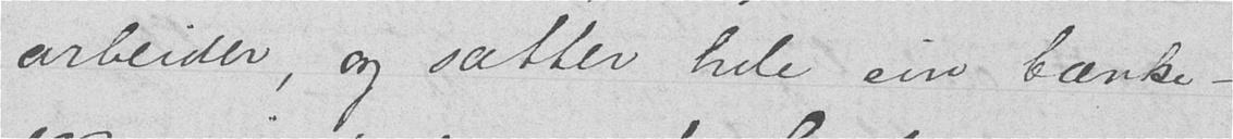arbeider, og sætter hele sin tanke-
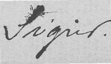Sigrid.
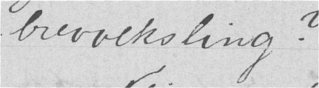brevveksling?
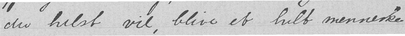du helst vil, blive et helt menneske
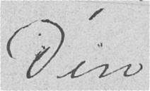Din
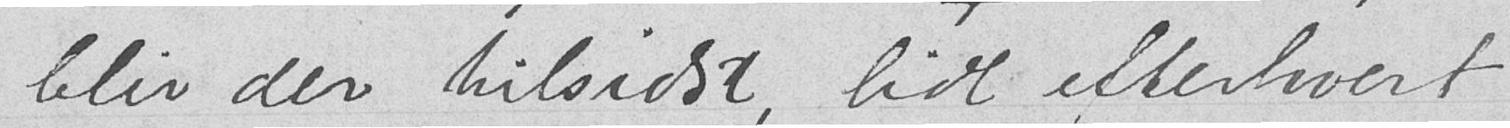blir der tilsidst, lidt efterhvert
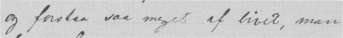og forstaa saa meget af livet, man
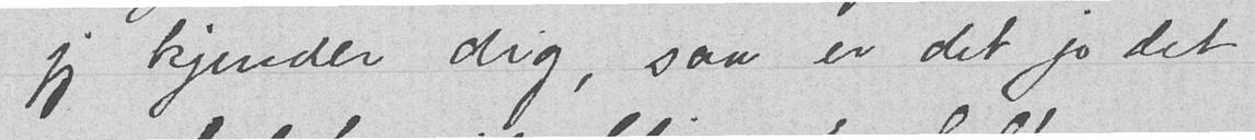jeg kjender dig, saa er det jo det
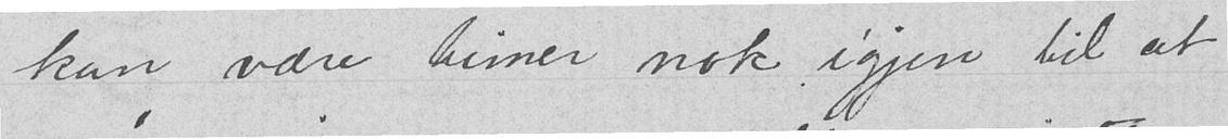kan være timer nok igjen til at
